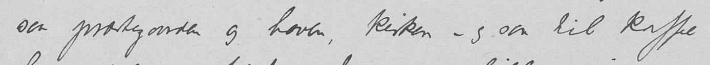saa præstegaarden og haven, kirken – og saa til kaffe
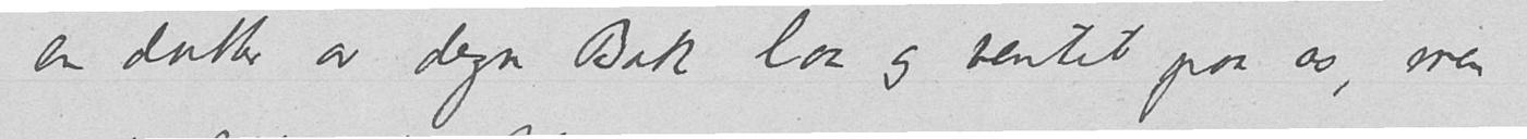en datter av degn Bak laa og ventet paa os, men
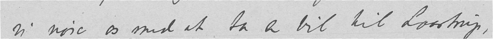vi nøie os med at ta en bil til Laastrup,
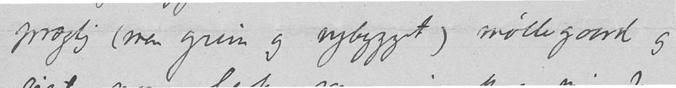prægtig (men grim og nybygget) møllegaard og
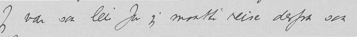Jeg var saa lei for jeg maatte reise derfra saa
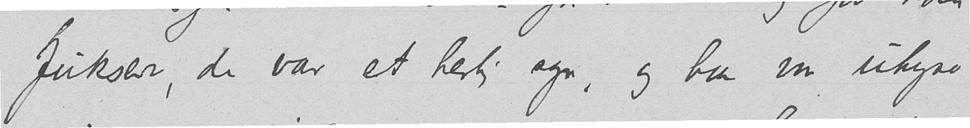fukser, de var et herlig syn, og han var uhyre
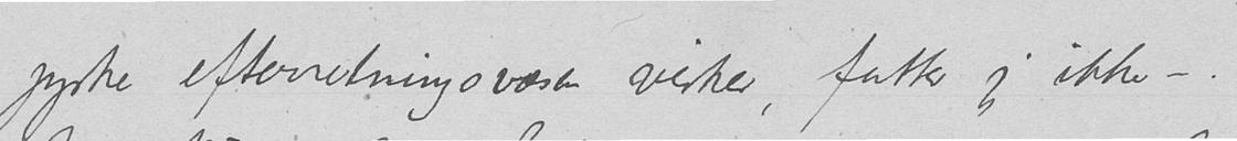jyske efterretningsvæsen virker, fatter jeg ikke –.
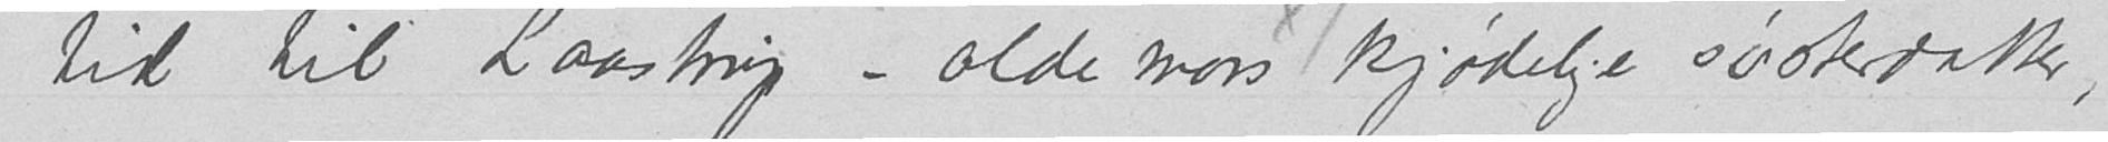tid til Laastrup – oldemors kjødelige søsterdatter,
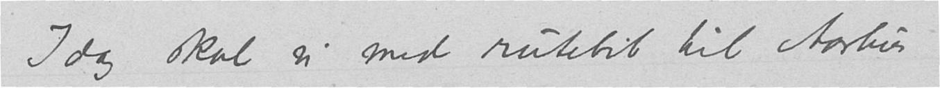I dag skal vi med rutebil til Aarhus
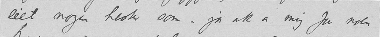eiet nogen hester som – ja ak a mig for noen
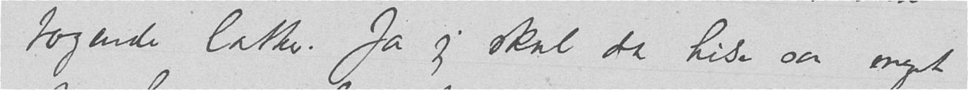tagende latter. Ja jeg skal da hilse saa meget
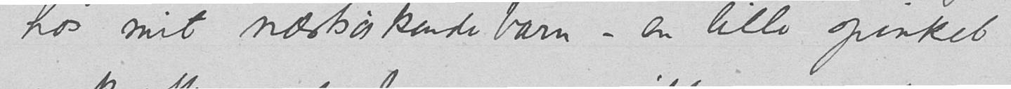hos mit næstsøskendebarn – en lille spinkel
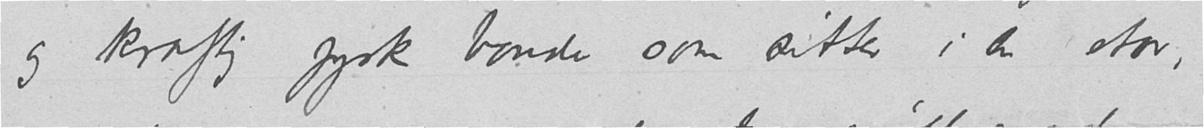og kraftig jysk bonde som sitter i en stor,
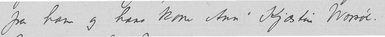fra ham og hans kone Ann Kjærstin Worsøe.
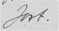fort.
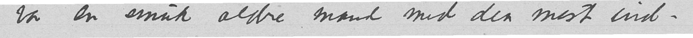var en smuk ældre mand med den mest ind-
